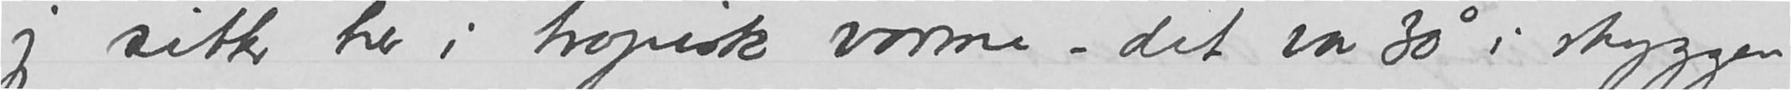Jeg sitter her i tropisk varme – det var 30° i skyggen
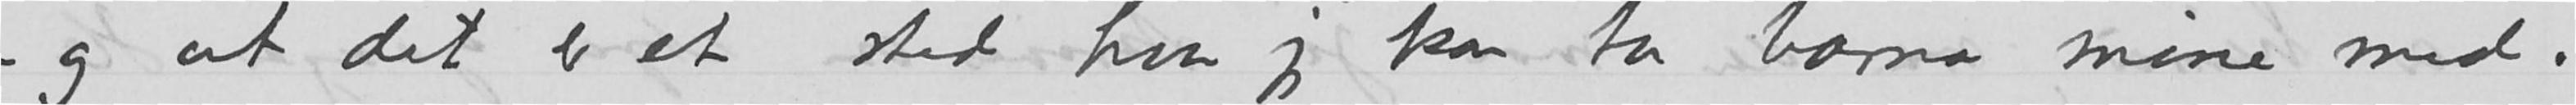– og at det er et sted hvor jeg kan ta barna mine med.
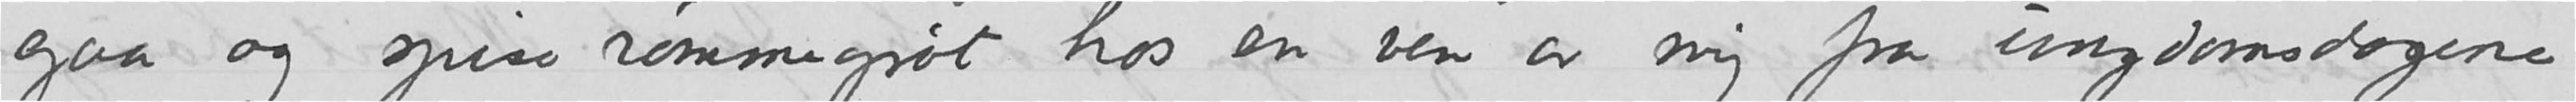gaa og spise rømmegrøt hos en ven av mig fra ungdomsdagene
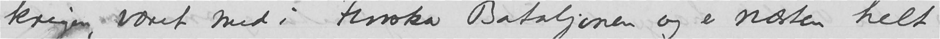krigen, været med i Finska Bataljonen og er næsten helt
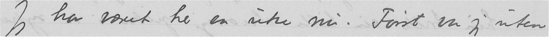Jeg har været her en uke nu. Først var jeg uten
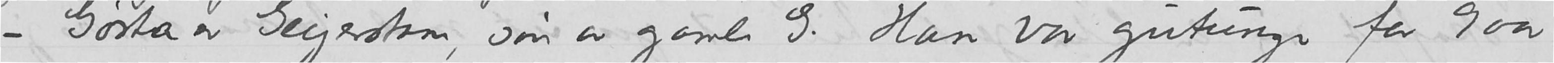– Gøsta av Geijerstam, søn av gamle G. Han var gutunge for 9 aar
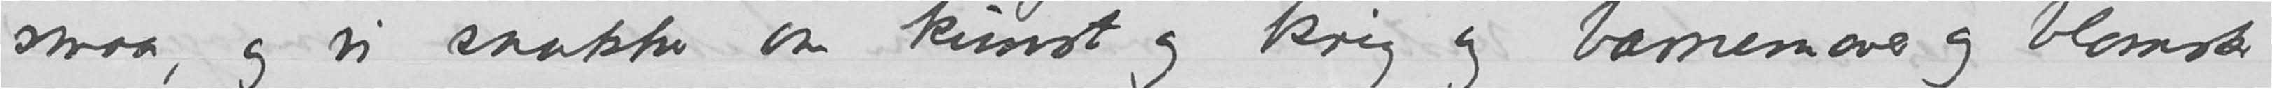smaa, og vi snakker om kunst og krig og barnene vore og blomster
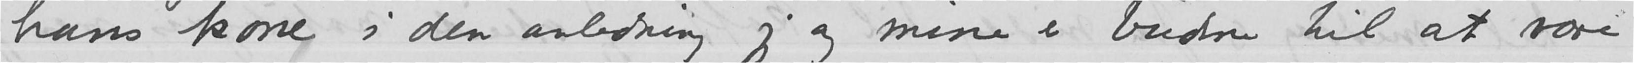hans kone i den anledning jeg og mine er budne til at være
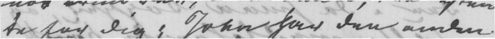te for Dig; John har den anden
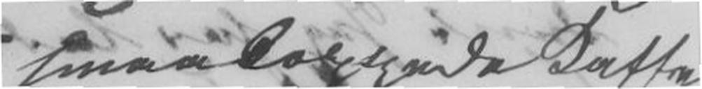smaakoppede kaffe-
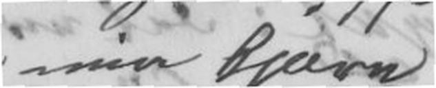min kjære
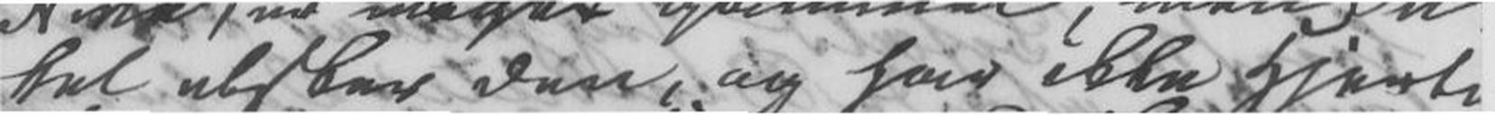kel elsker den, og har ikke hjerte
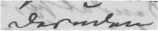der uden
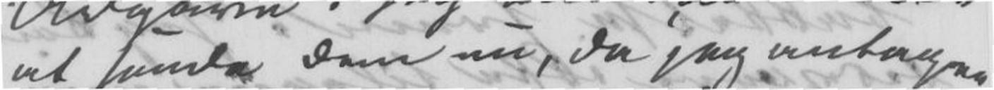at sende dem nu, da jeg antager
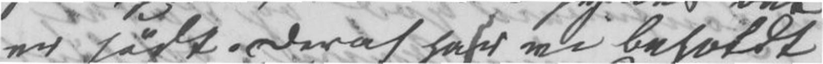er sødt deraf har vi beholdt
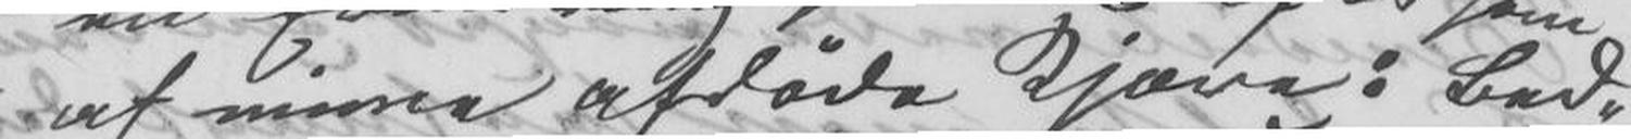af mine afdøde Kjære: bed-
